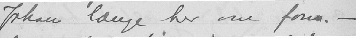Johan længe her om form. -
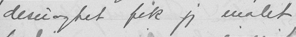desuagtet fik jeg malet
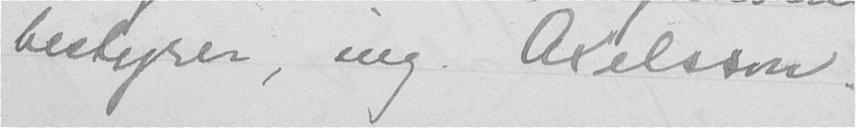bestyrer, ing. Axelsson.
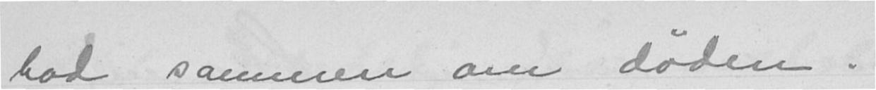bad sammen om døden.
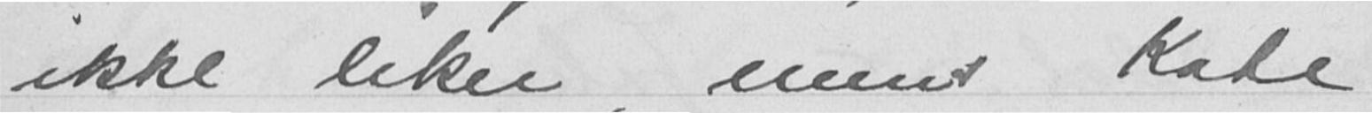ikke liker, men Kate
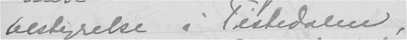bestyrelse i Tistedalen,
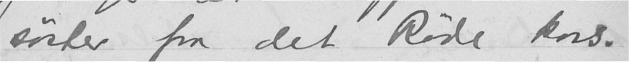søster fra det Røde kors.
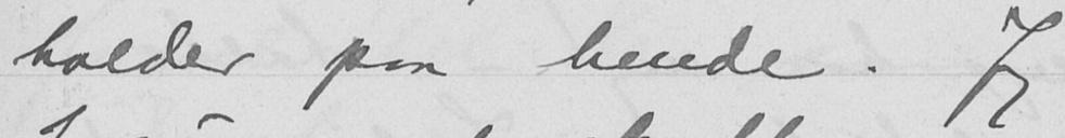holder paa hende. Jeg
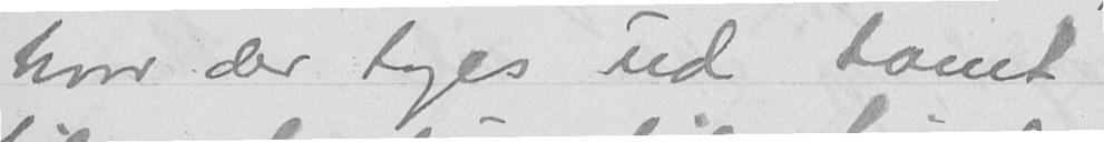hvor der tages ud tomt
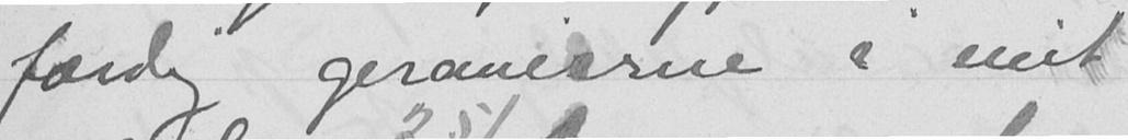færdig geranierne i mit
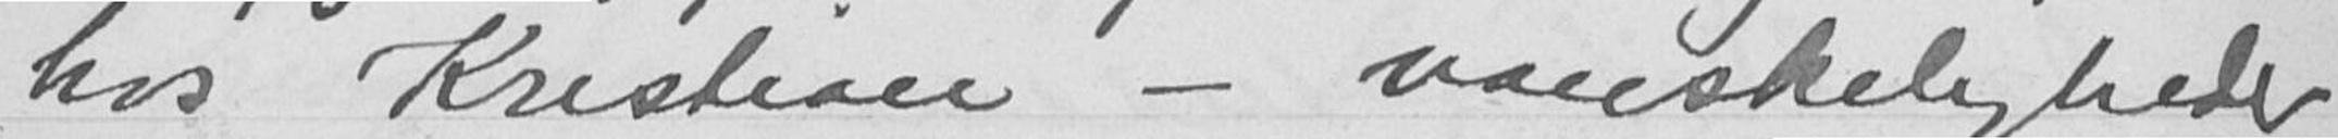hos Kristian - vanskeligheder
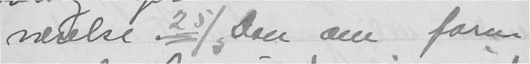værelse. 25/5 Don om form
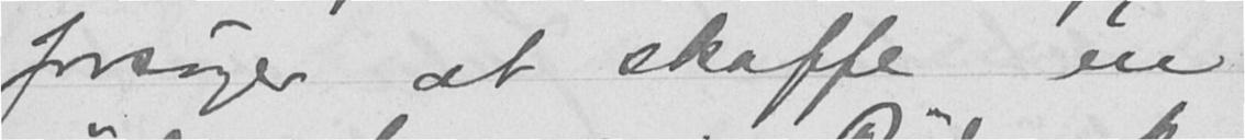forsøger at skaffe en
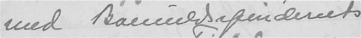med Bomuldspinderiets
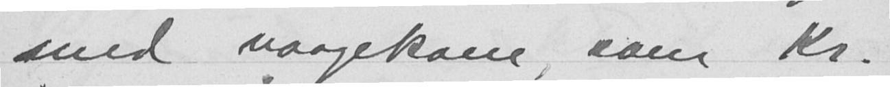med vaagekone, som Kr.
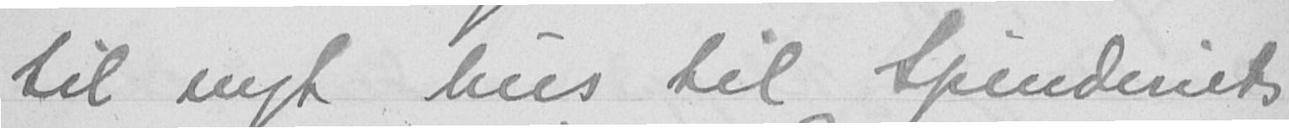til nyt hus til Spinderiets
